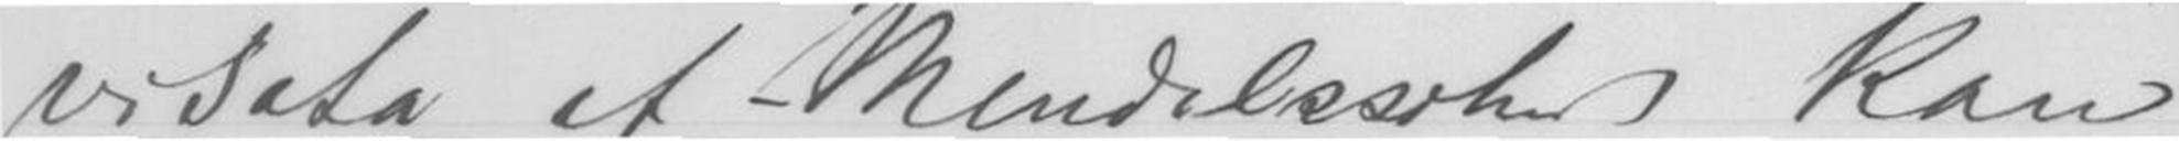visata af Mendelssohns kan
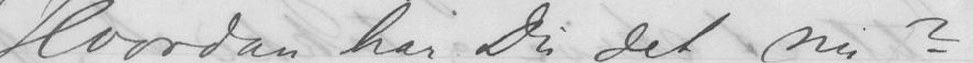Hvordan har Du det nu?
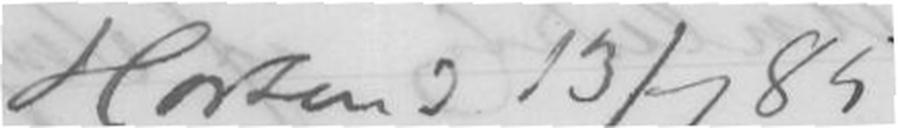Horten 13/7 85
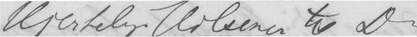Hjertelig Hilsener til Dig.
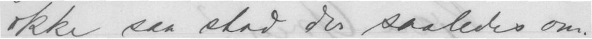ikke saa stod du saaledes om-
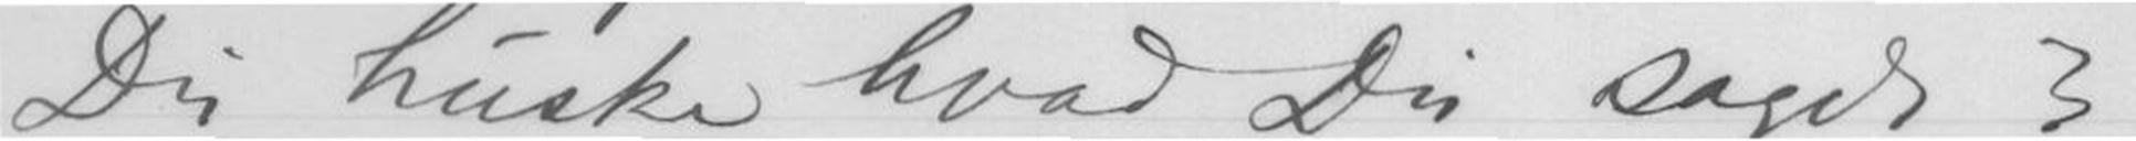Du huske hvad Du sagde 3
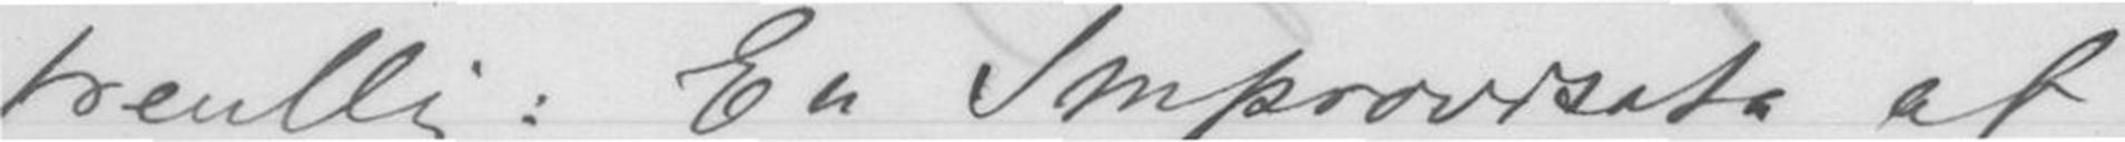trentlig: En Improsata af
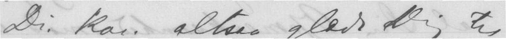Du kan altsaa glæde Dig til
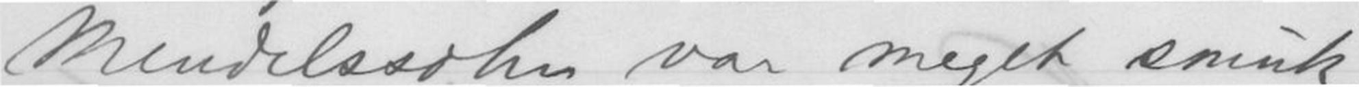Mendelssohn var meget smuk,
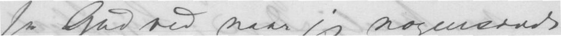Ja Gud ved naar jeg nogensinde
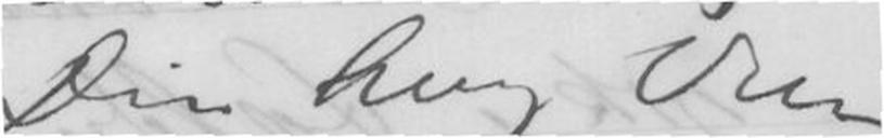Din heng Ven
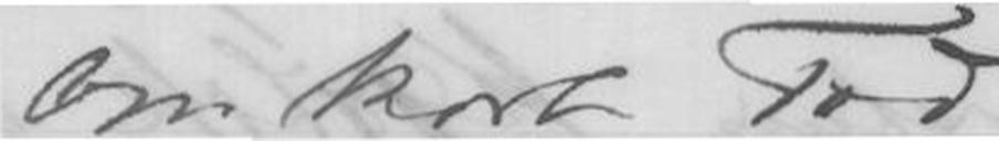om kort Tid.
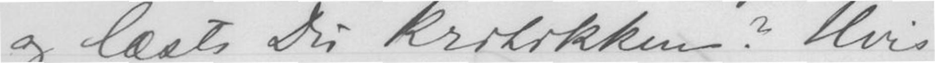og læste Du Kritikken? Hvis
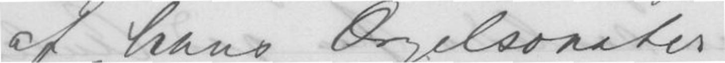af hans Orgelsonater.
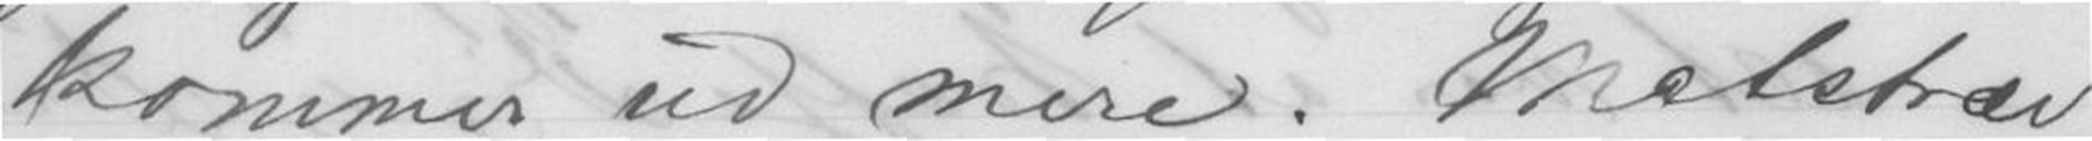kommer ud mere. Matstræv
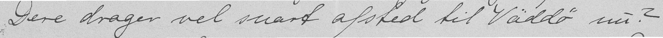Dere drager vel snart afsted til Väddø nu?
